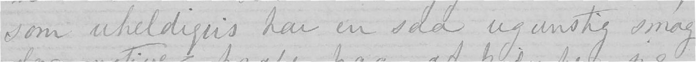som uheldigvis har en saa ugunstig smag
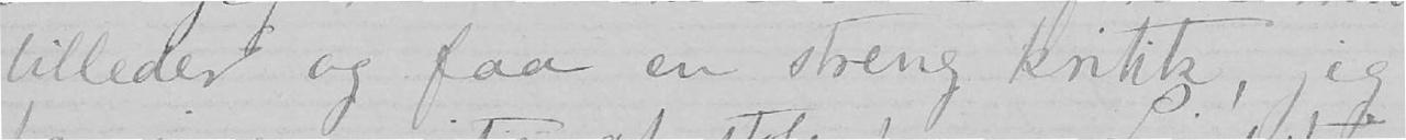billeder og faa en streng kritik, jeg
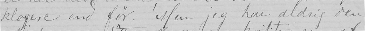klogere end før. Men jeg har aldrig den
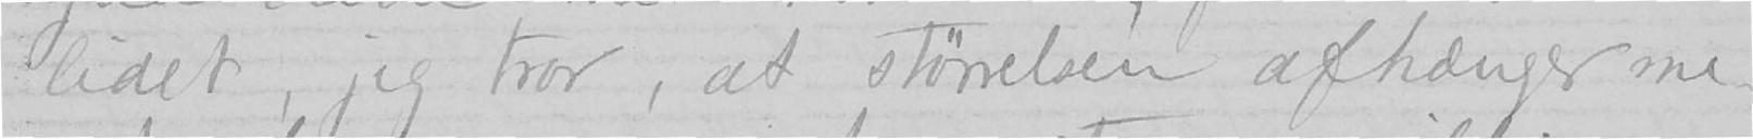lidet, jeg tror, at størrelsen afhænger me-
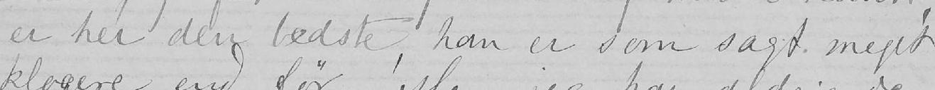er her den bedste, han er som sagt meget
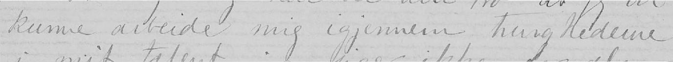kunne arbeide mig igjennem tunghederne
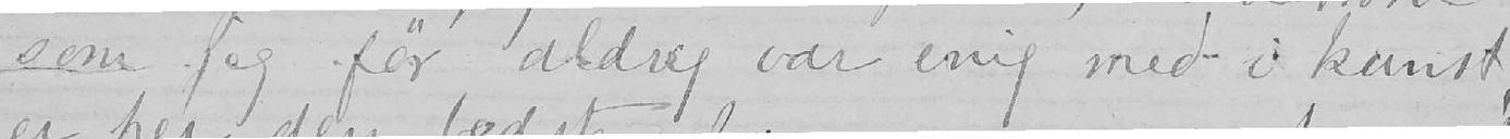som jeg før aldrig var enig med i kunst,
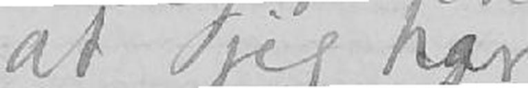at jeg har
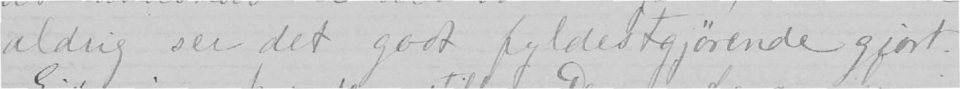aldrig ser det godt fyldestgjørende gjort.
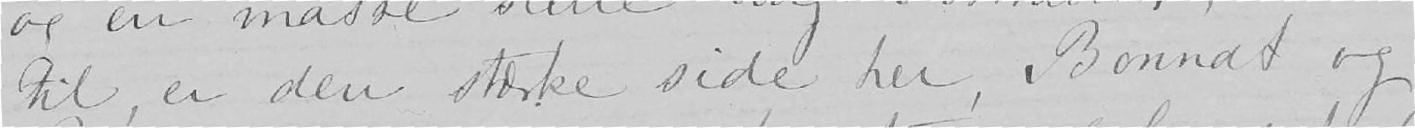til, er den stærke side her, Bonnat og
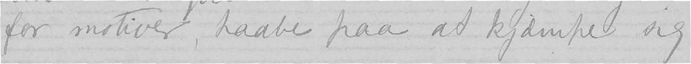for motiver, haabe paa at kjæmpe sig
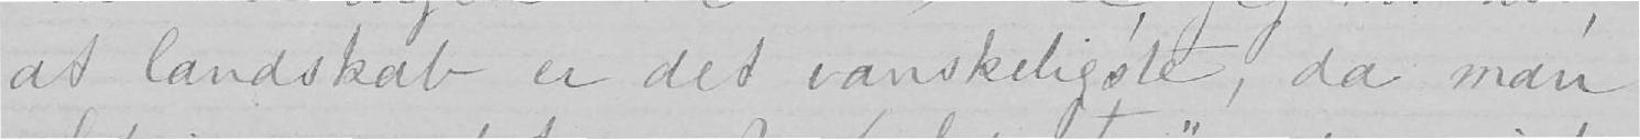at landskab er det vanskeligste, da man
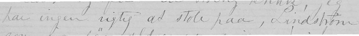har ingen rigtig at stole paa, Lindstrøm
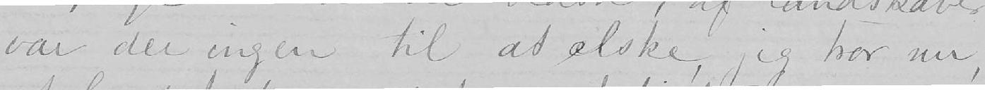var der ingen til at elske, jeg tror nu,
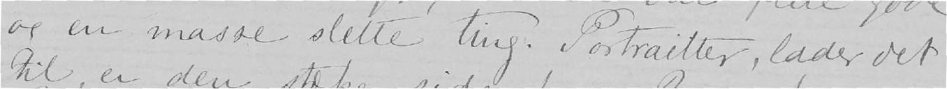og en masse slette ting. Portraitter, lader det
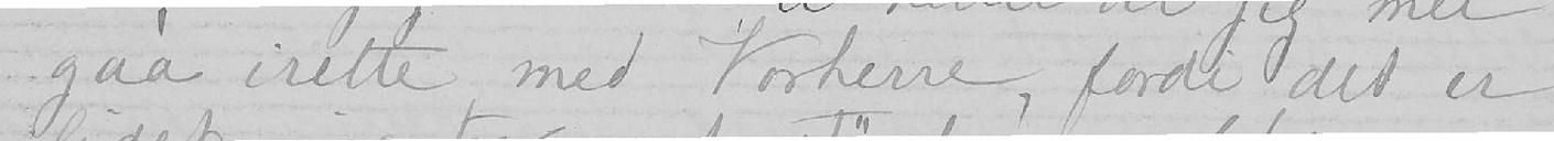gaa irette med Vorherre, fordi det er
